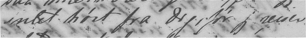intet hørt fra Dig, før jeg reiser
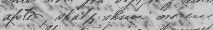afsted, skal jeg skrive nøiere
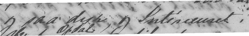og saa dyr, og Intérieuret i
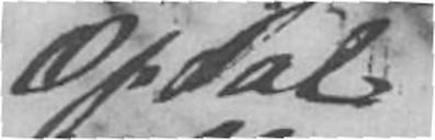Opdal
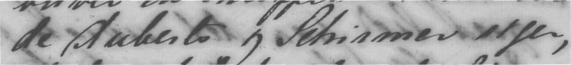de Auberts og Schirmer siger,
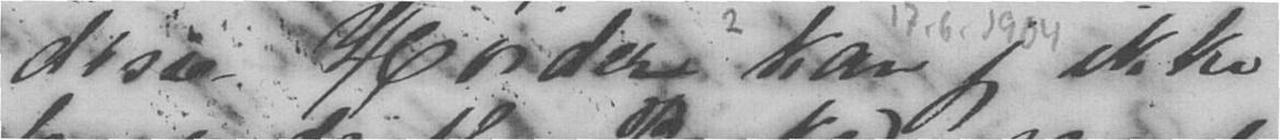disse Høider kan jeg ikke
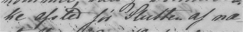ke afsted før Slutten af næ-
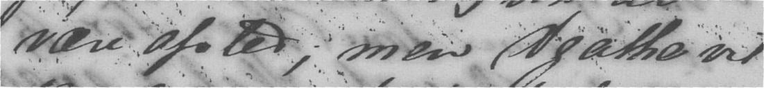være afsted, men Agathe vil
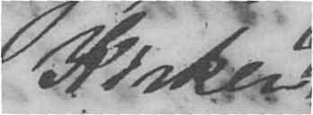Kirken
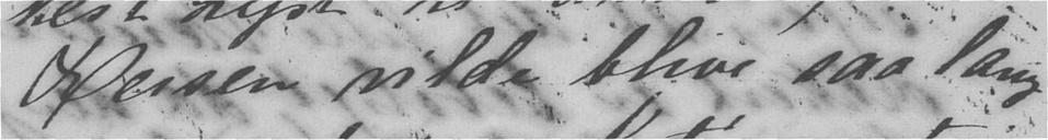Reisen vilde blive saa lang
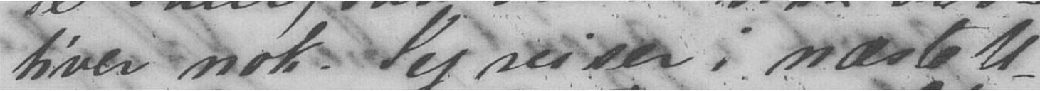tiver nok. Jeg reiser i næste U-
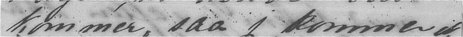kommer, saa jeg kommer ik-
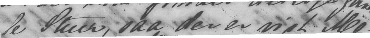le Stuer, saa der er vist Mo-
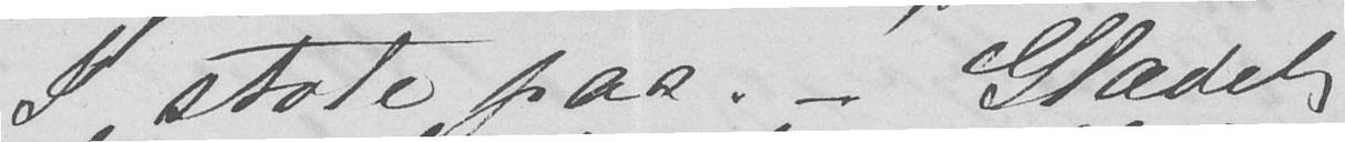I stole paa. - Glædelig
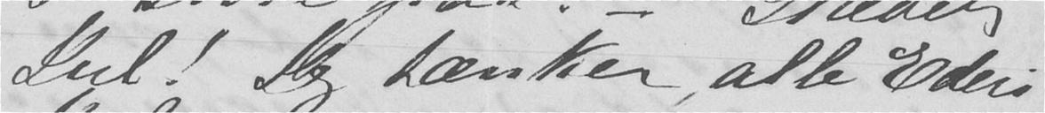Jul! Jeg tænker, alle Eders
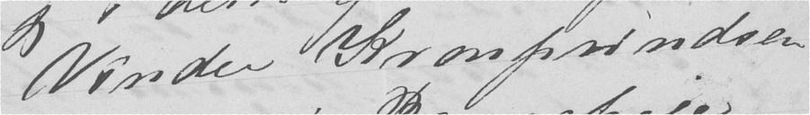Vindu Kronprindsen
